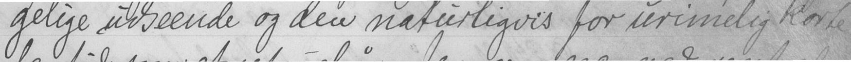gelige udseende og den naturligvis for urimelig korte
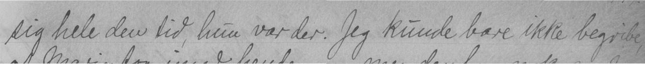sig hele den tid, hun var der. Jeg kunde bare ikke begribe,
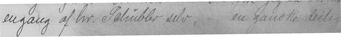engang af hr. Schubber selv, - en ganske deilig
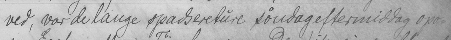ved, var de lange spadsereture søndag eftermiddag opo-
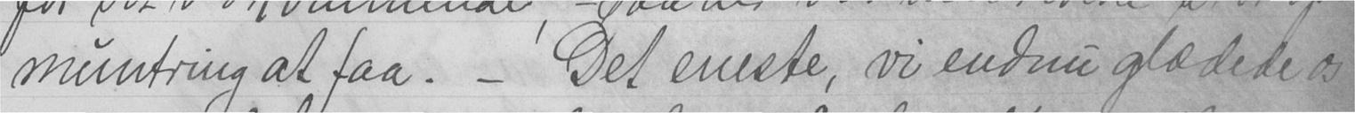muntring at faa. - Det eneste, vi endnu glædede os
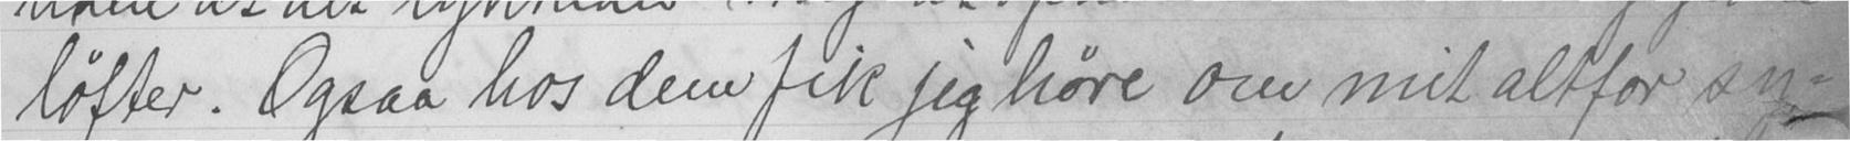løfter. Ogsaa hos dem fik jeg høre om mit altfor sy-
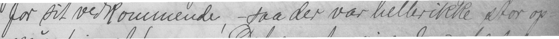for sit vedkommende, - saa der var hellerikke stor op-
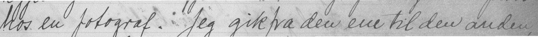hos en fotograf. Jeg gik fra den ene til den anden,
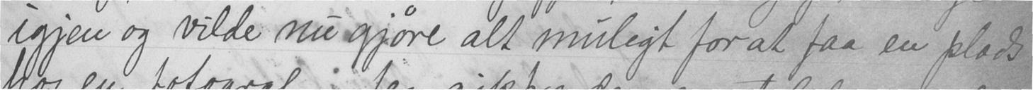igjen og vilde nu gjøre alt muligt for at faa en plads
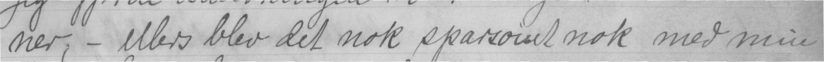ner, - ellers blev det nok sparsomt nok med min
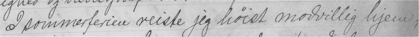I sommerferien reiste jeg høist modvillig hjem;
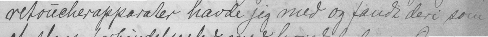retoucherapparater havde jeg med og fandt deri som
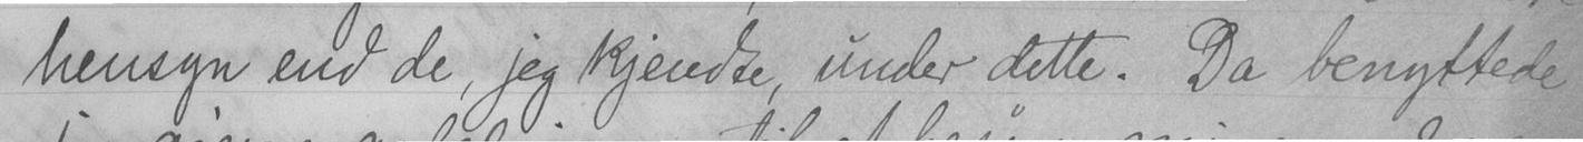hensyn end de, jeg kjendte, under dette. Da benyttede
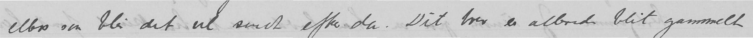ellers saa blir det vel sendt efter da.  Dit brev er allerede blit gammelt
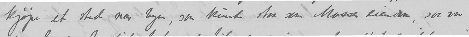kjøpe et sted nær byen, som kunde staa som Mosses eiendom, saa var
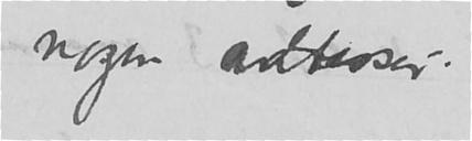nogen addresser.
